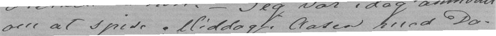om at spise Middag i Aasen med Da-
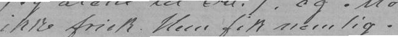ikke frisk. Hun fik nemlig.
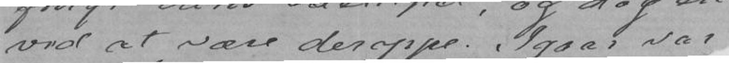ved at være deroppe. Igaar var
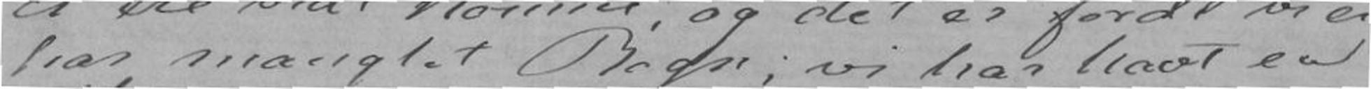har manglet Regn; vi har havt en
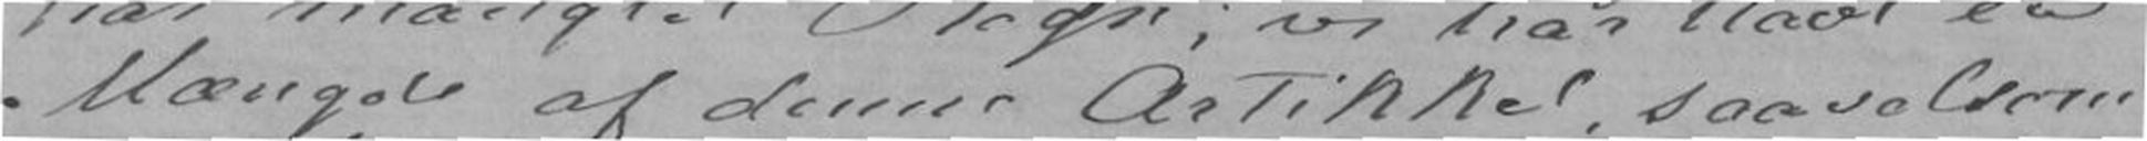Mængde af denne Artikkel, saavelsom
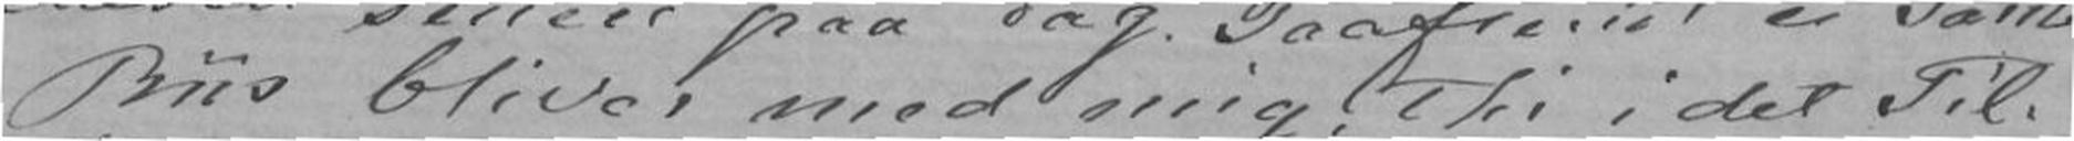Riis bliver med mig, thi i det Til-
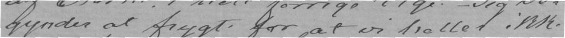gynder at frygte for at vi heller ikke
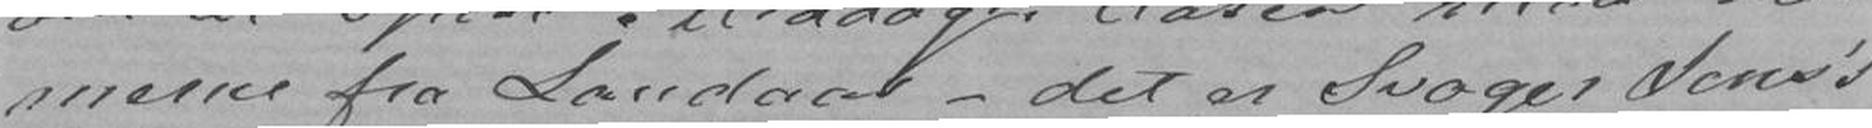merne fra Landaas - det er Svoger Jens's
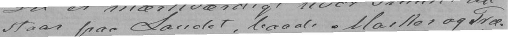staar paa Landet baade Marker og Træ-
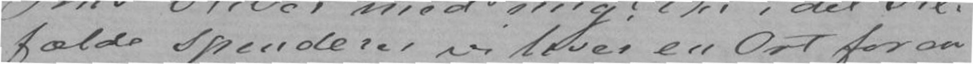fælde spenderer vi hver en Ort for en
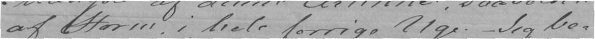af Storm i hele forrige Uge. - Jeg be-
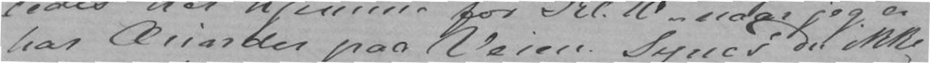har Ærinder paa Veien. Synes Du ikke
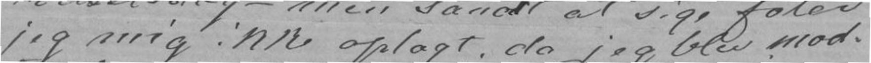jeg mig ikke oplagt, da jeg blev mod-
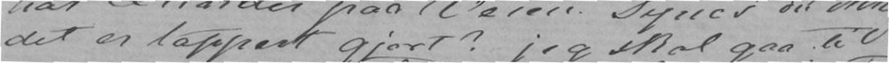det er tappert gjort? jeg skal gaa til
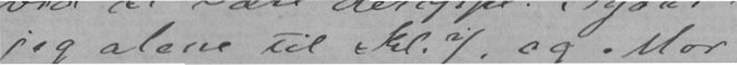jeg alene til Kl.7. og Mor
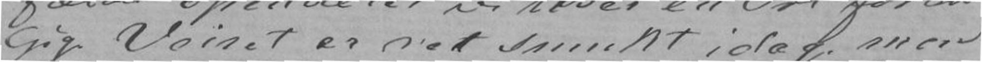Gig. Veiret er ret smukt idag, men
